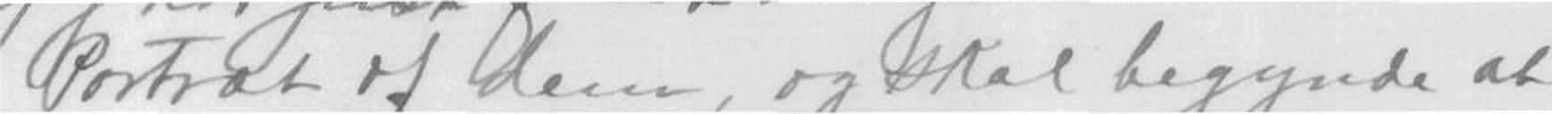Portræt of dem, og skal begynde at
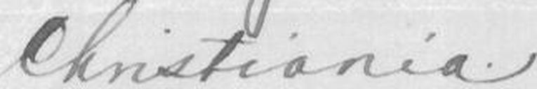Christiania
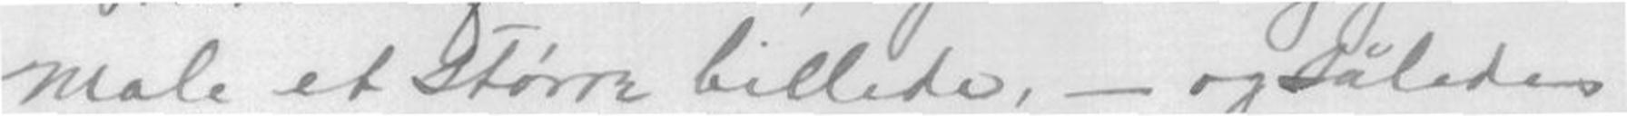male et større billede, - og således
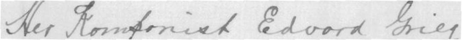Her Komponist Edvard Grieg
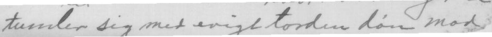tumler sig med evige torden døn mod
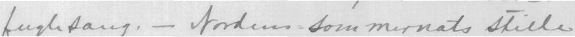fuglesang. - Nordens som mernats stille
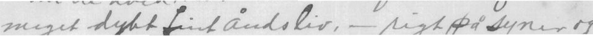meget dybt fint Åndsliv, - rigt på syner og
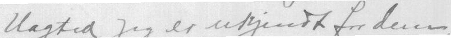Uagted jeg er ukjendt for dem,
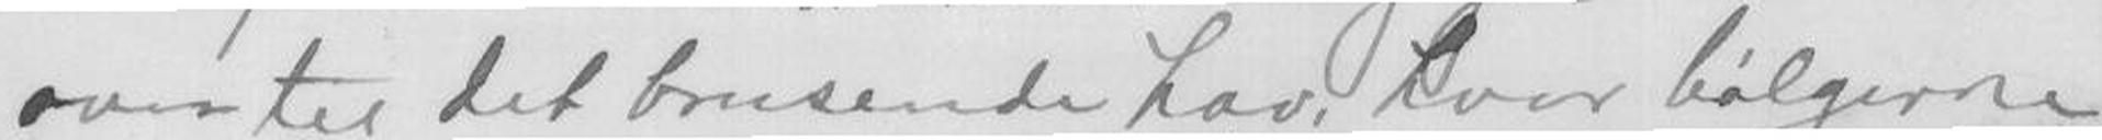over til det brusende hav, hvor bølgerne
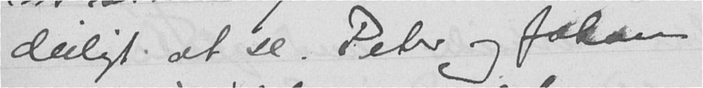deiligt at se. Peter og Johan
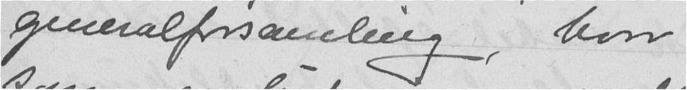generalforsamling, hvor
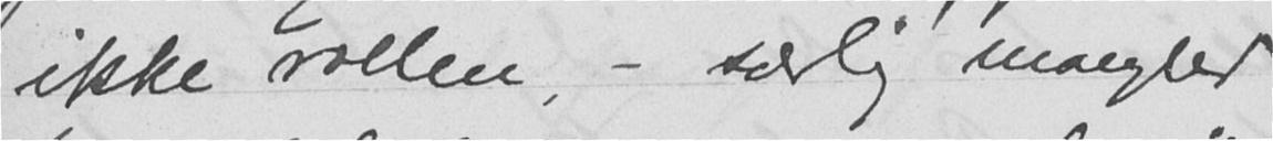ikke rollen, - særlig mangled
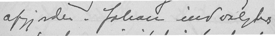afgjordes. Johan indvalgtes
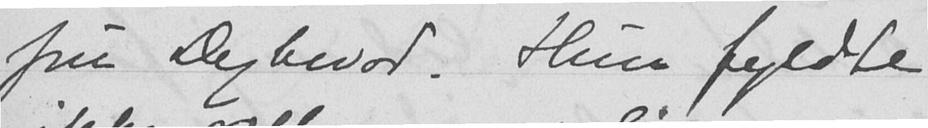fru Dybwad. Hun fyldte
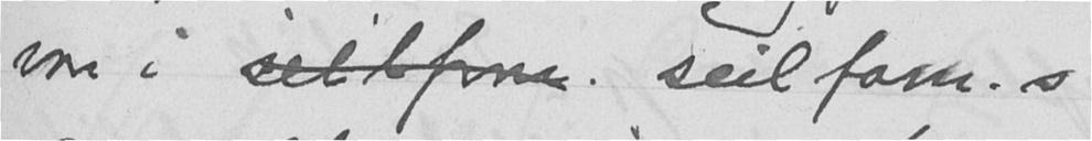var i sellforn. seilforn.s
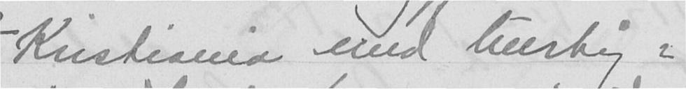Kristiania med hurtig-
